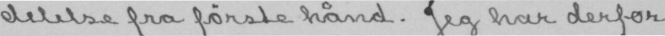delelse fra første hånd. Jeg har derfor
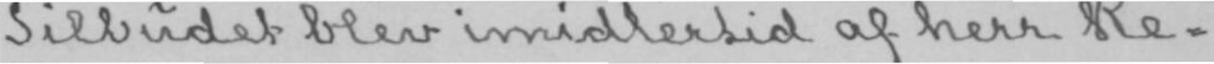Tilbudet blev imidlertid af herr Re-
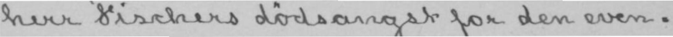herr Fischers dødsangst for den even-
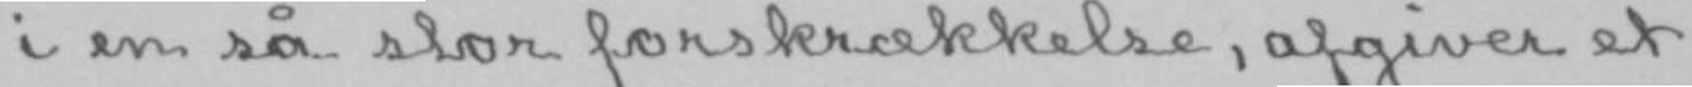i en så stor forskrækkelse, afgiver et
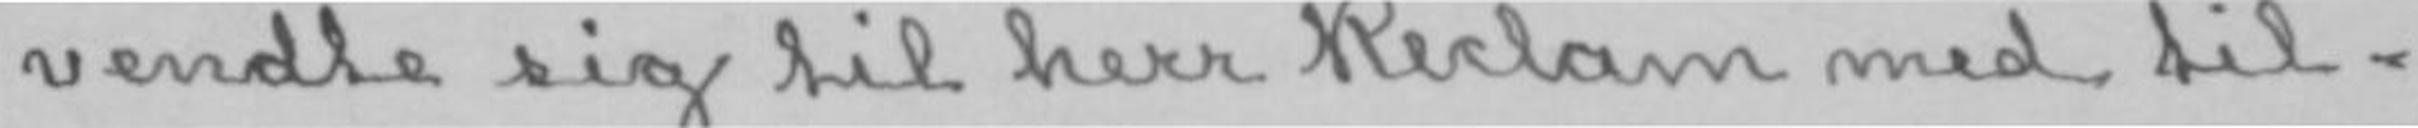vendte sig til herr Reclam med til-
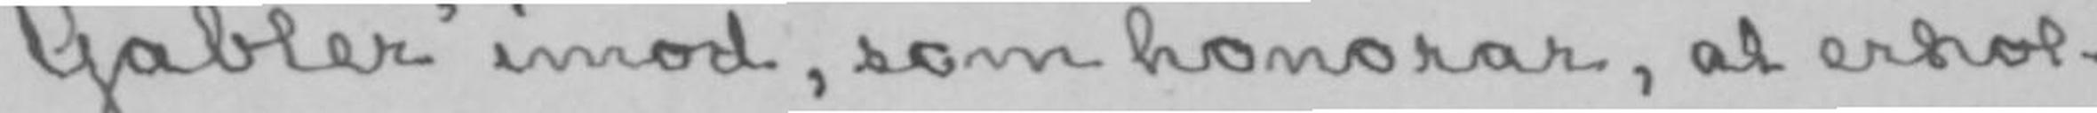Gabler» imod, som honorar, at erhol-
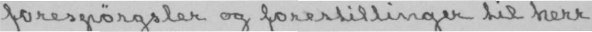forespørgsler og forestillinger til herr
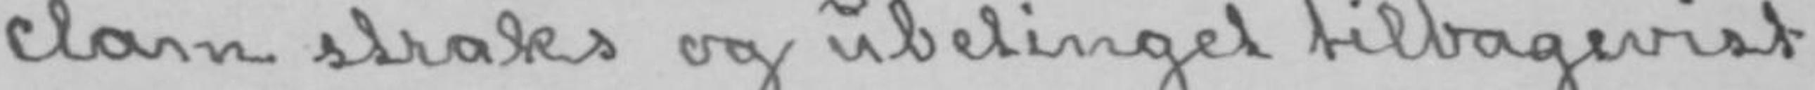clam straks og ubetinget tilbagevist.
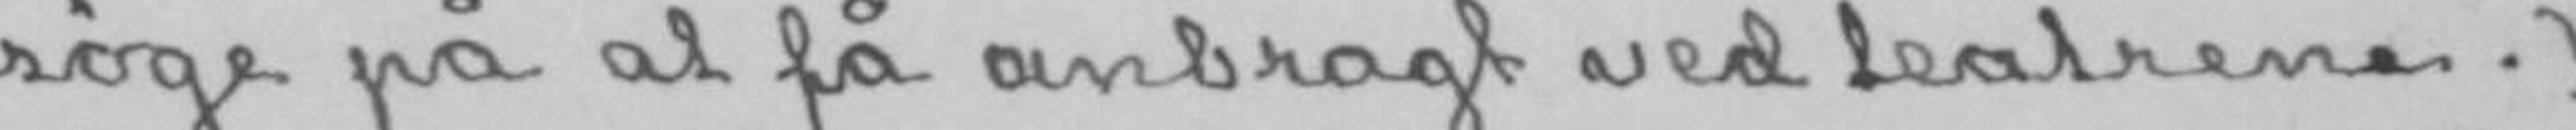søge på at få anbragt ved teatrene.)
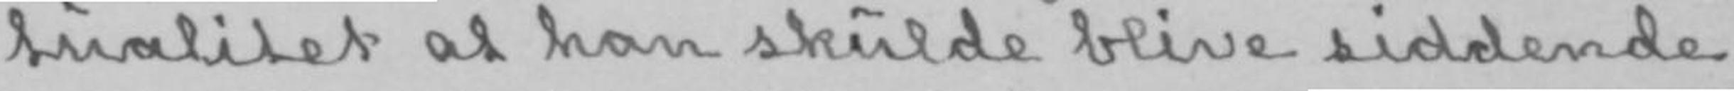tualitet at han skulde blive siddende
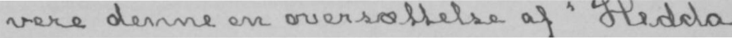vere denne en oversættelse af «Hedda
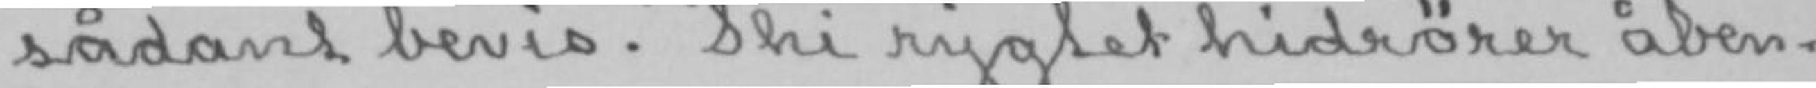sådant bevis. Thi rygtet hidrører åben-
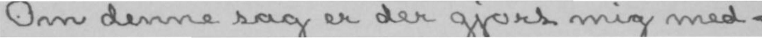Om denne sag er der gjort mig med-
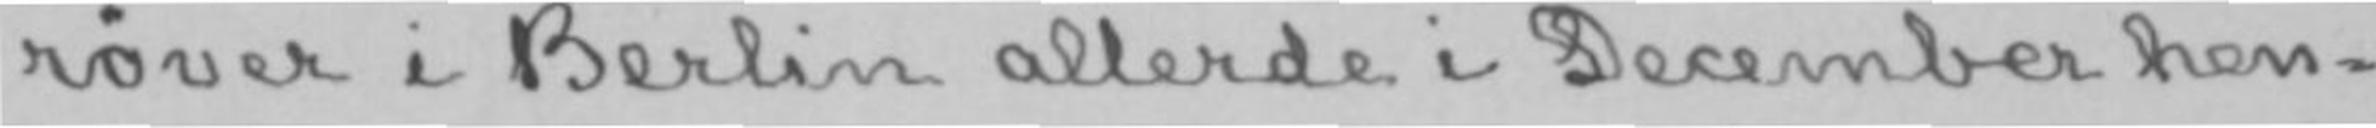røver i Berlin allerde i December hen-
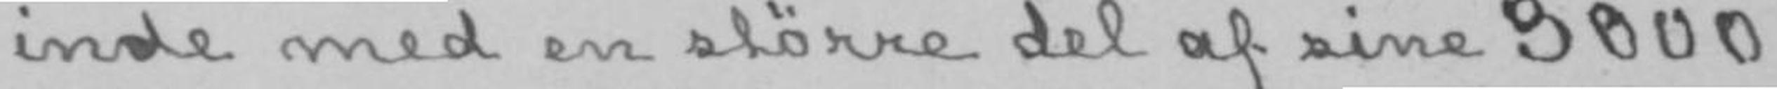inde med en større del af sine 3000
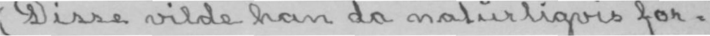(Disse vilde han da naturligvis for-
